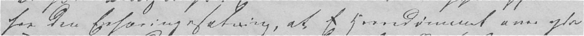fra den Erfaringssætning, at  Herredømmet over ydre
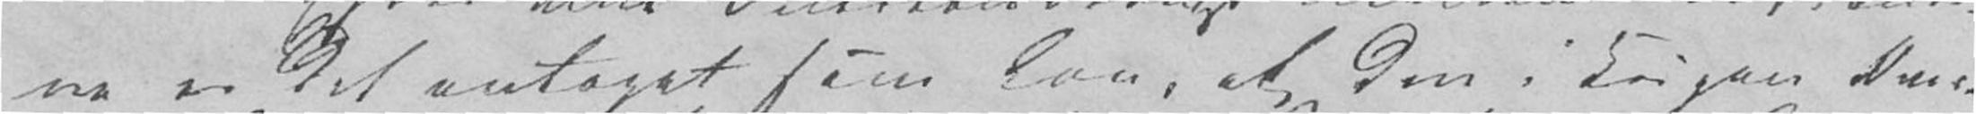ne er det antaget som Lov, at den i Krigen Over-
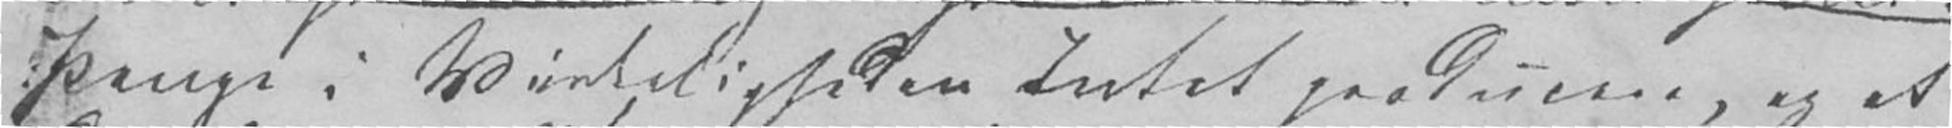Penge i Virkeligheden Intet producere, og at
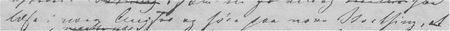læse i vore Aviser og høre paa vore Storthing, at
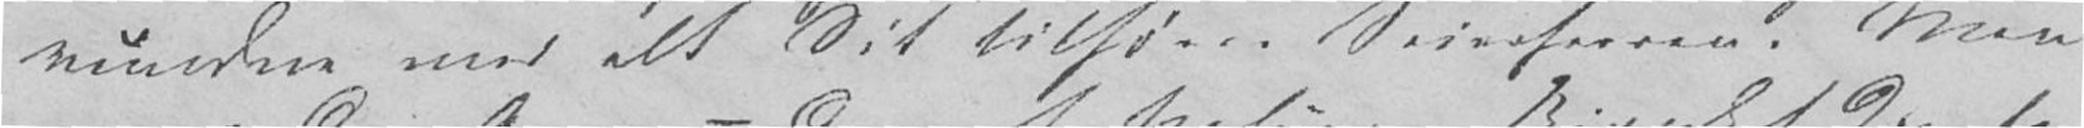vundne med alt sit tilhører Seierherreren. Men
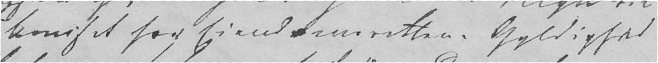Beviset for Eiendomsrettens Gyldighed
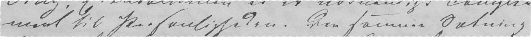ment til Personligheden. Den samme Sætning
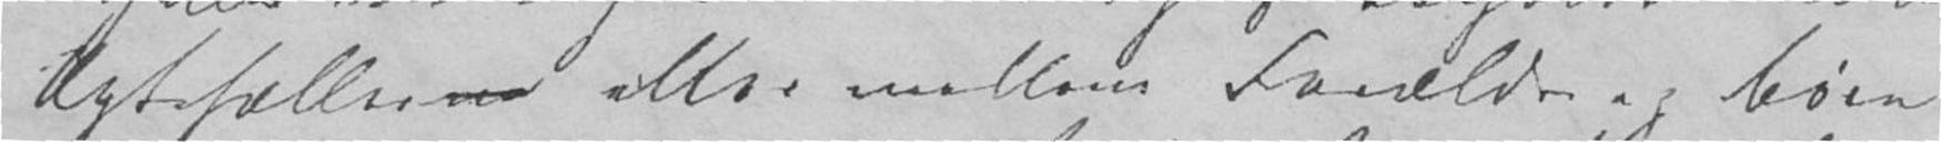Ægtefællerne eller mellem Forældre og Børn
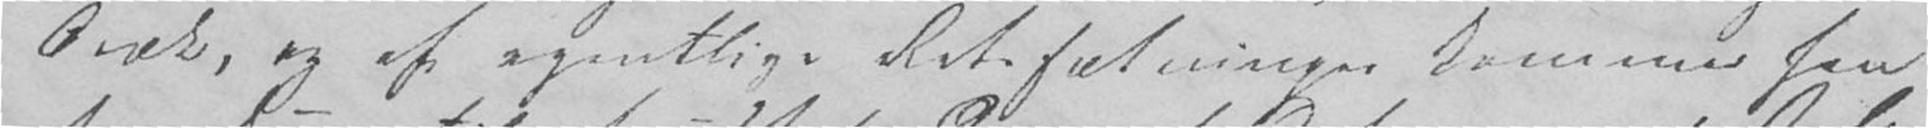Træk, og af egentlige Retssætninger kommer han
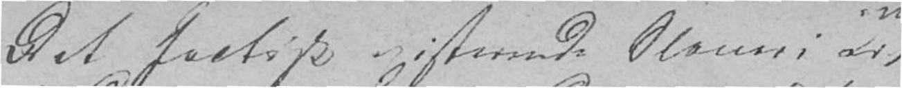Det factisk existerende Slaveri er
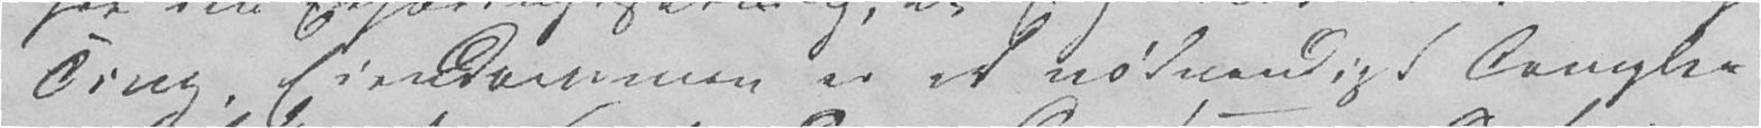Ting, Eiendommen er et nødvendigt Comple-
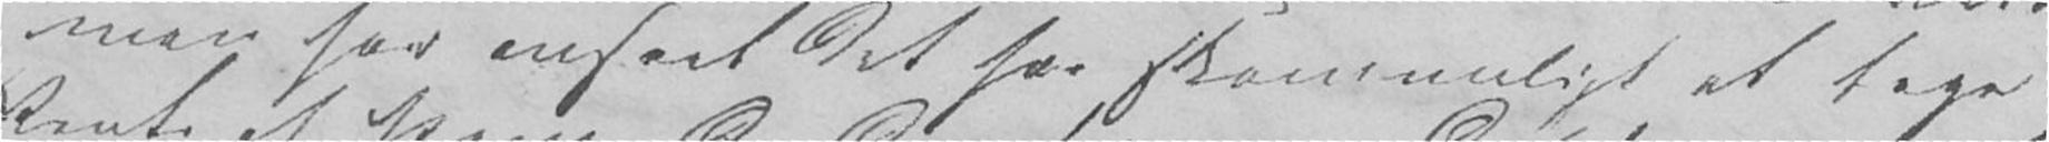man har anseet det for skammeligt at tage
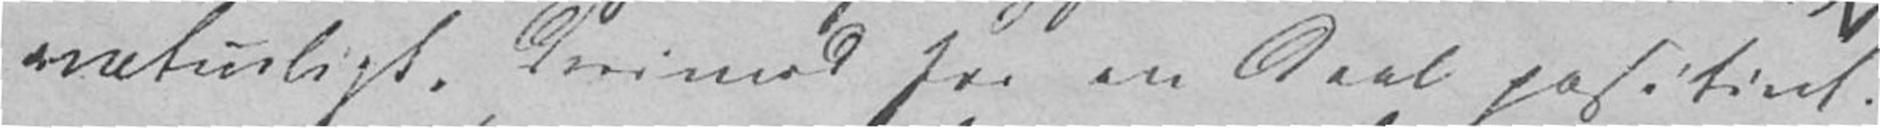naturligt. Derimod for en Deel positivt.
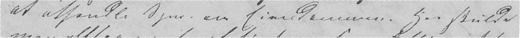at afhandle Spm. om Eiendommen. Her skulde
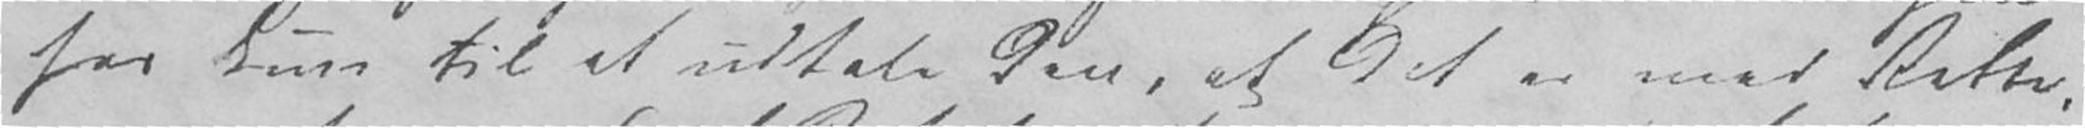her kun til at udtale den, at det er med Rette,
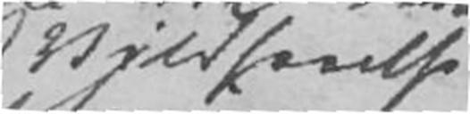Vildfarelse
